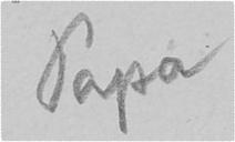Papa
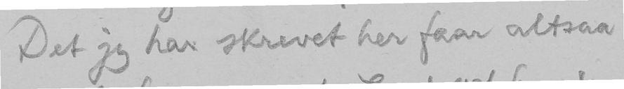Det jeg har skrevet her faar altsaa
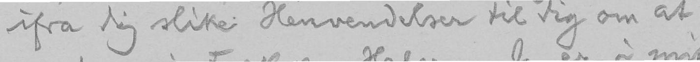ifra dig slike Henvendelser til dig om at
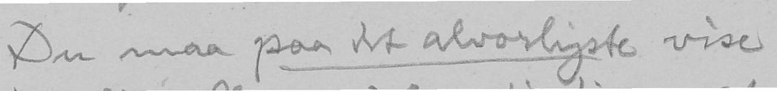Du maa paa det alvorligste vise
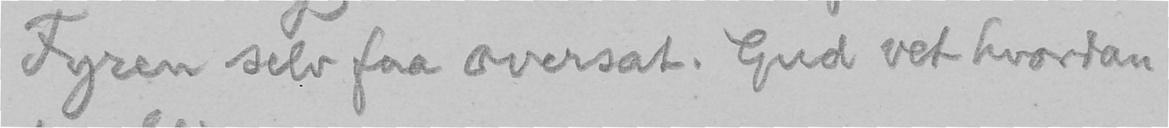Fyren selv faa oversat. Gud vet hvordan
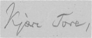Kjære Tore,
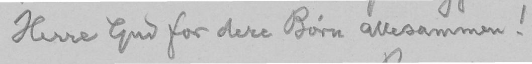Herre Gud for dere Børn allesammen!
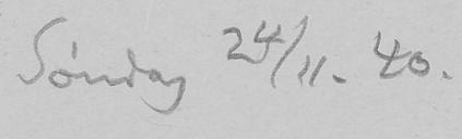Søndag 24/11. 40.
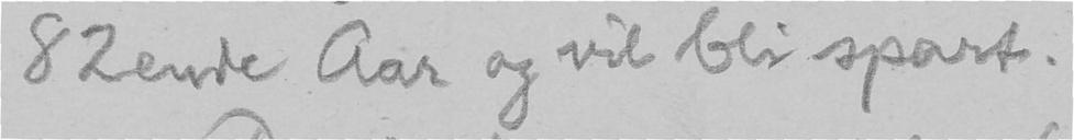82ende Aar og vil bli spart.
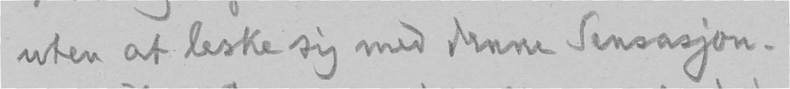uten at leske sig med denne Sensasjon.
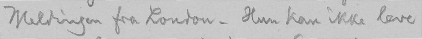Meldingen fra London. Hun kan ikke leve
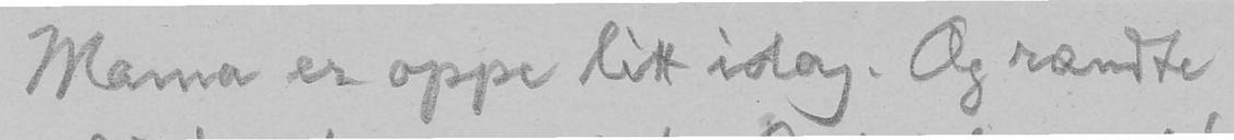Mama er oppe litt idag. Og rændte
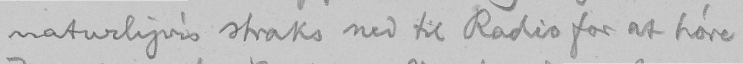naturligvis straks ned til Radio for at høre
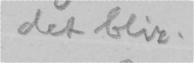det blir.
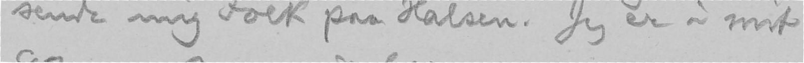sende mig Folk paa Halsen. Jeg er i mit
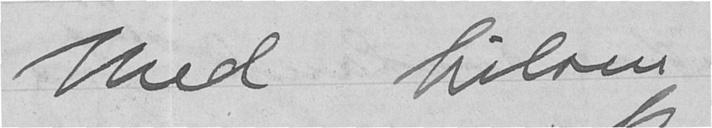Med hilsen
